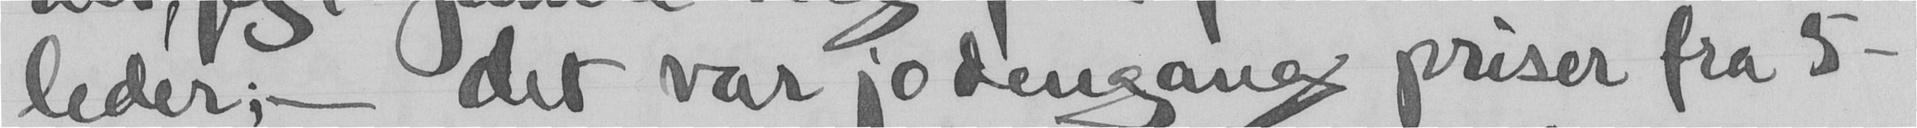leder; - det var jo dengang priser fra 5 -
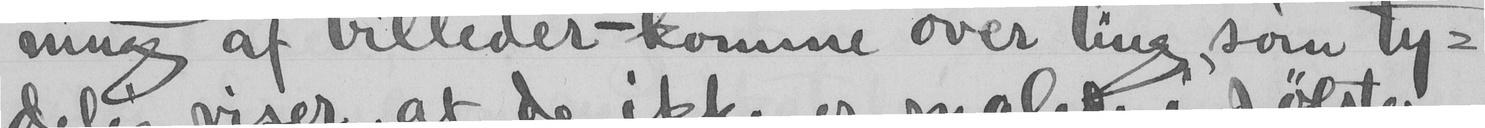ning af billeder - komme over ting, som ty-
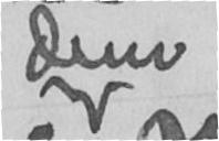dem
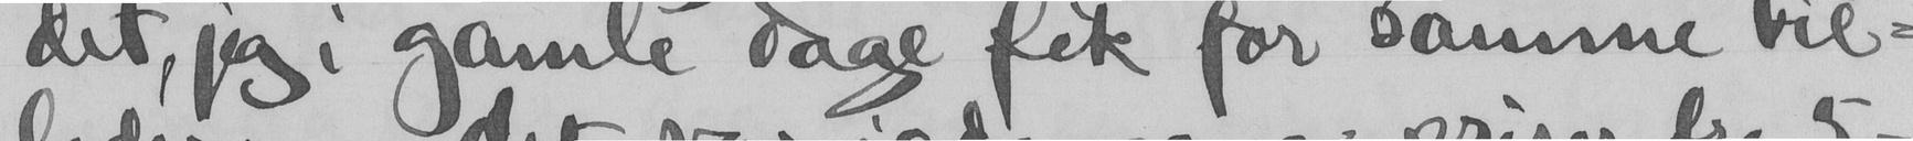det, jeg i gamle dage fik for samme bil-
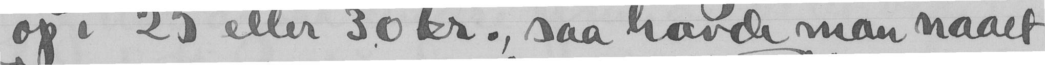op i 25 eller 30 kr., saa havde man naaet
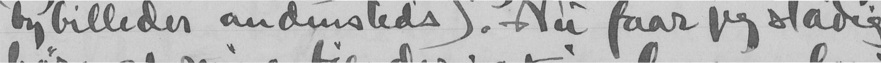by billeder andensteds). Nu faar jeg stadig
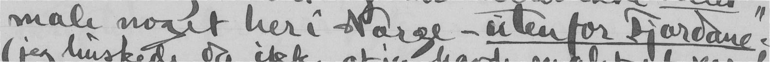male noget her i Norge - utenfor Fjordane";
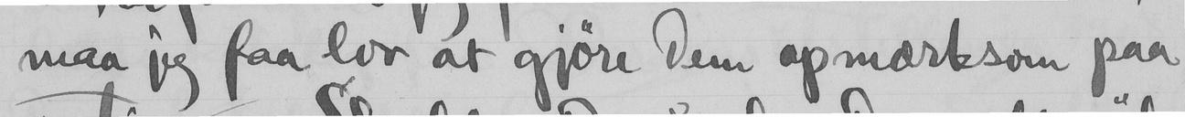maa jeg faa lov at gjøre Dem opmærksom paa
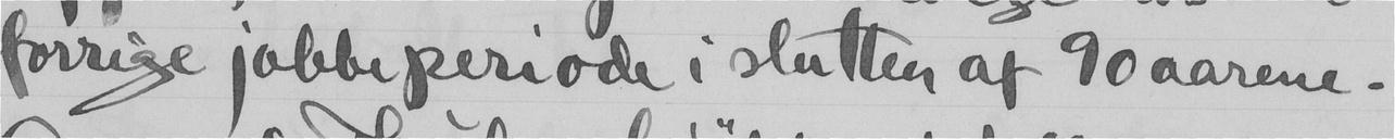forrige jobbeperiode i slutten af 90 aarene.-
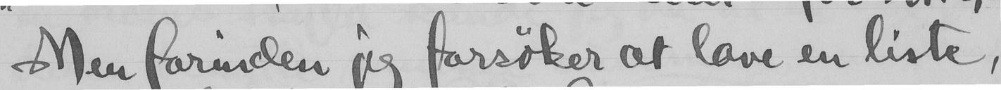Men forinden jeg forsøker at lave en liste,
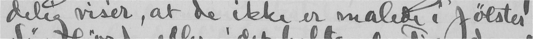delig viser, at de ikke er malede i Jølster
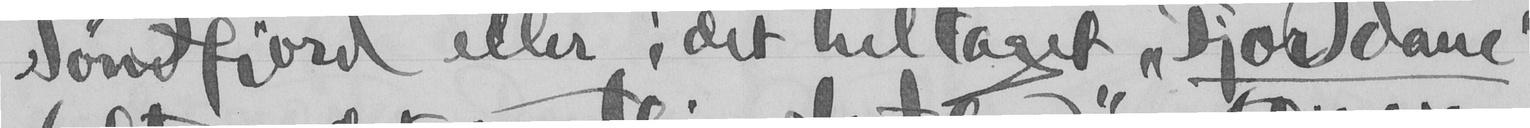Søndfjord eller idet heltaget "Fjordane"
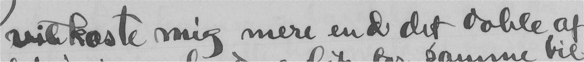vil koste mig mere end det doble af
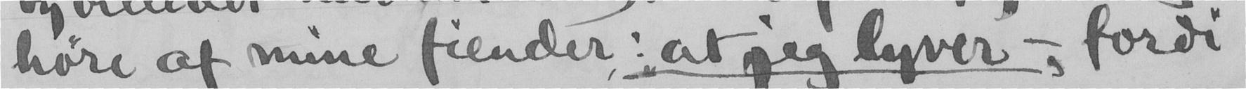høre af mine fiender: at jeg lyver - fordi
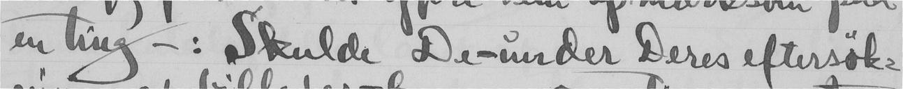en ting -: skulde De-under Deres eftersøk-
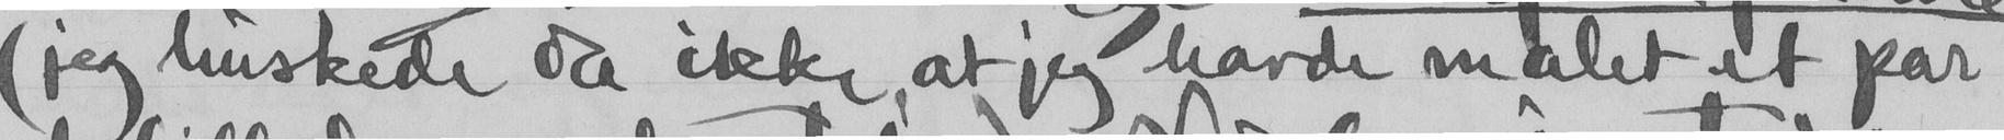(jeg huskede da ikke, at jeg havde malet et par
